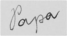Papa
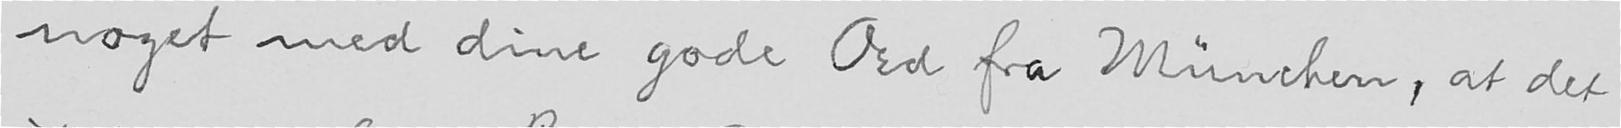noget med dine gode Ord fra München, at det
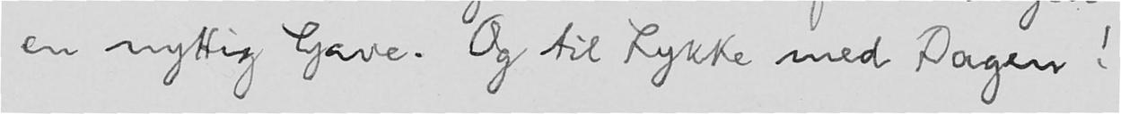en nyttig Gave. Og til Lykke med Dagen!
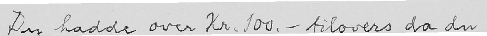Du hadde over Kr. 100. - tilovers da du
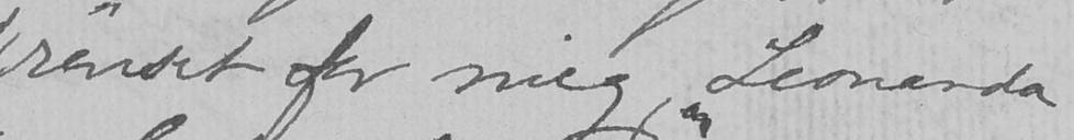rænset for mig, Leonarda
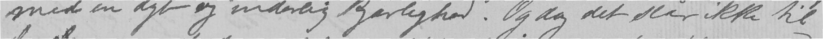med en dyb og inderlig kjærlighed. Og dog det slår ikke til
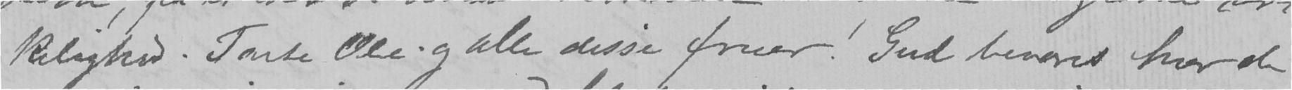kelighed. Tante Ole og alle disse fruer! Gud bevares hvor de
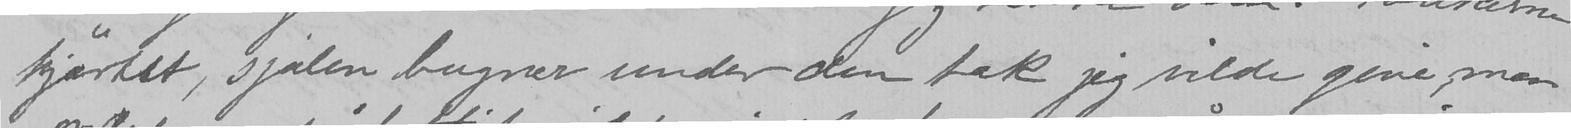hjærtet, sjælen bugner under den tak jeg vilde give, men
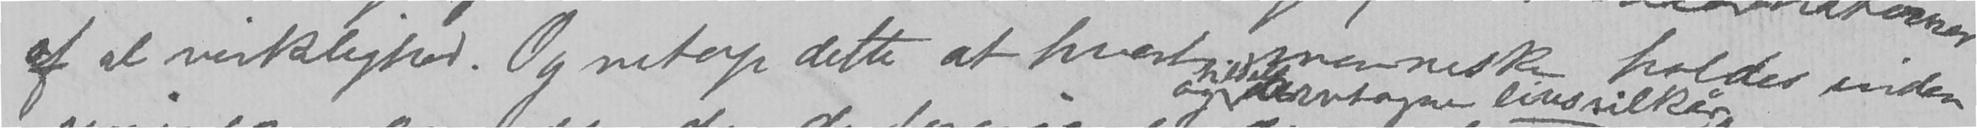af al virklighed. Og netop dette at hvert menneske holdes inden
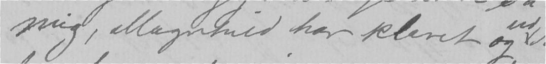mig, Magnhild har klaret og
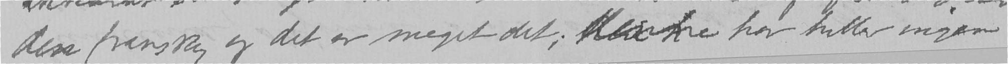den franske, og det er meget det; han har heller ingen
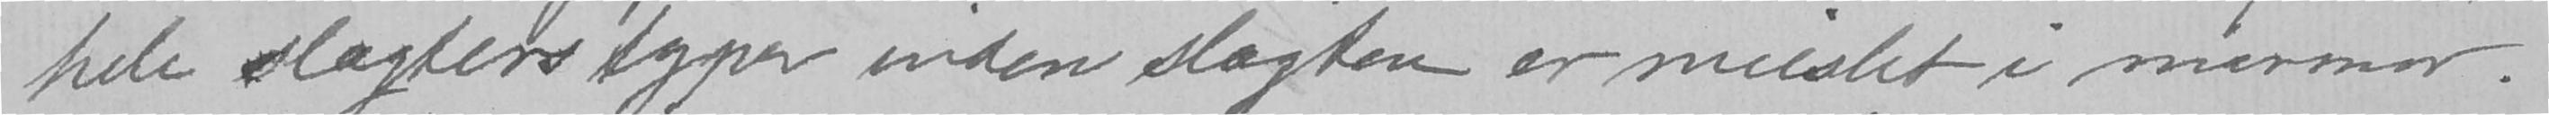hele slægtens typer inden slægten er meislet i marmor.
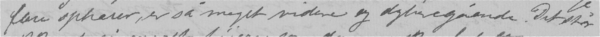flere sphærer, er så meget videre og dyberegående. Det står
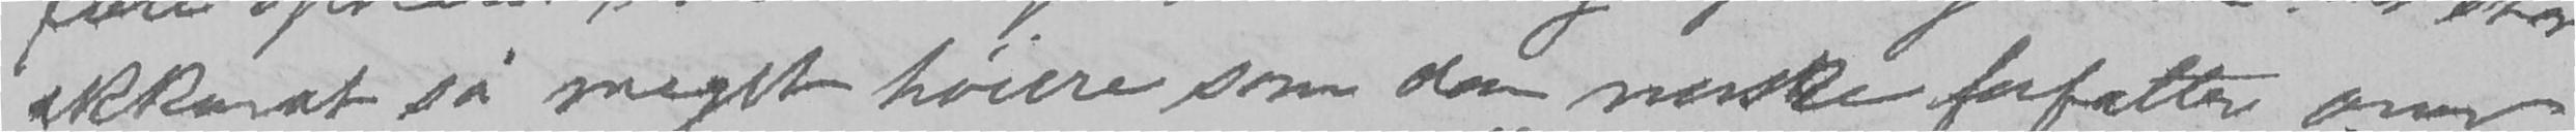akkurat så meget høiere som den norske forfatter over
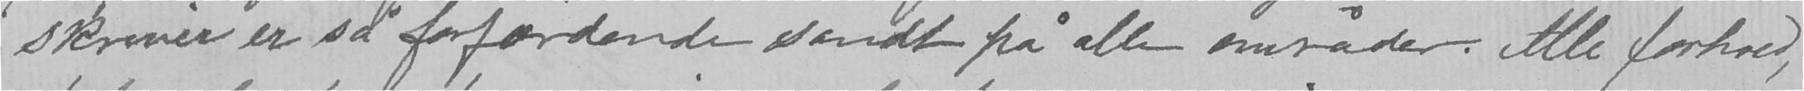skriver er så forfærdende sandt på alle områder. Alle forhold,
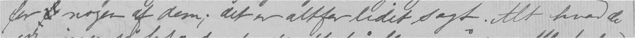for nogen af dem; det er altfor lidet sagt. Alt hvad de
